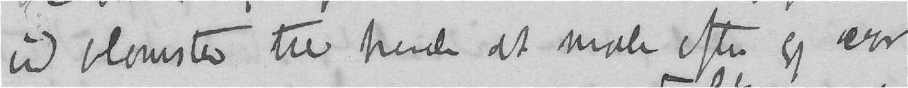ud blomster til hende at male efter og var
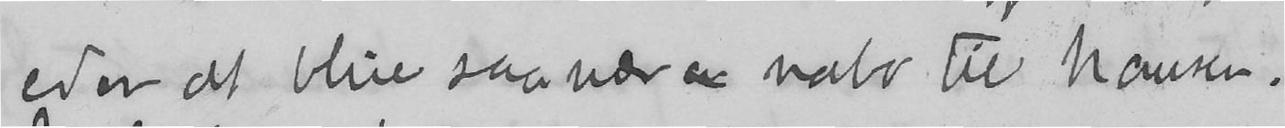eder at blive saanær en nabo til Nansen.
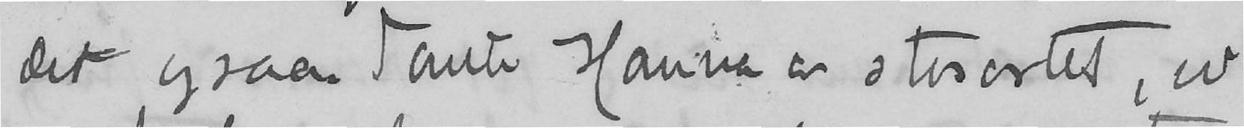det ogsaa. Tante Hanna er storartet, vi
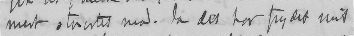mest storartet mod. Ja det har frydet mit
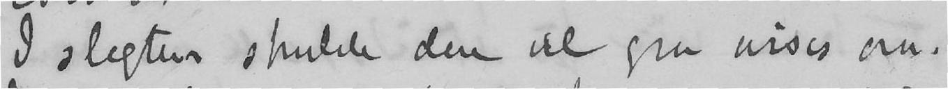I slegten skulde dere vel ogsaa vises om.
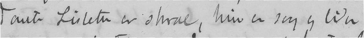Tante Lisbeth er skral, hun er svag og lider
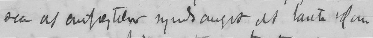saa af anfægtelser nyhedsangst at tante Han-
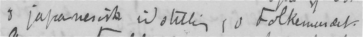i japanesisk udstilling og i Folkemusæet.
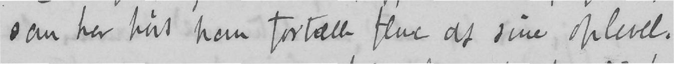som har hørt ham fortælle flere af sine oplevelser,
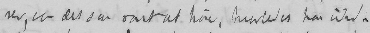var det saa rart at høre, hvorledes han undgik
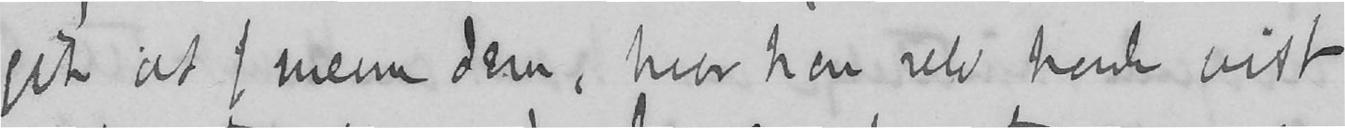at nævne dem, hvor han selv havde vist
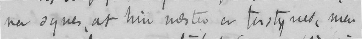na synes, at hun næsten er forstyrret, men
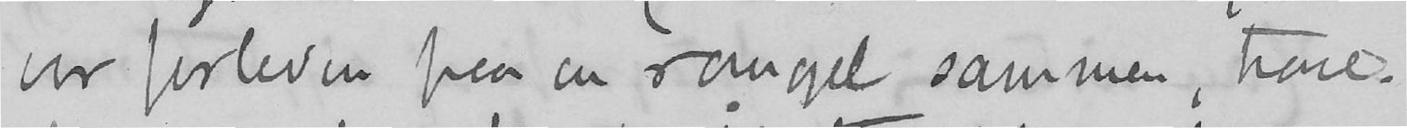var forleden paa en rangel sammen, ture-
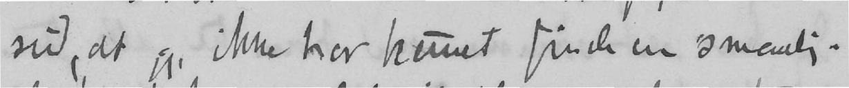sind, at jeg ikke har kunnet finde en smaalig
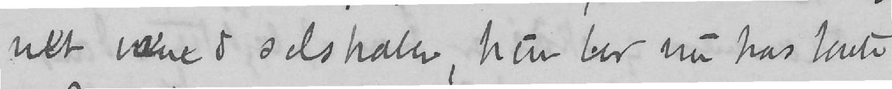net være i selskaber, hun bor nu hos tante
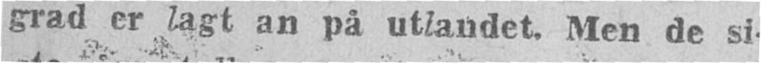grad er lagt an på utlandet. Men de si
